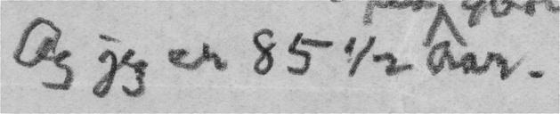Og jeg er 85½ Aar.
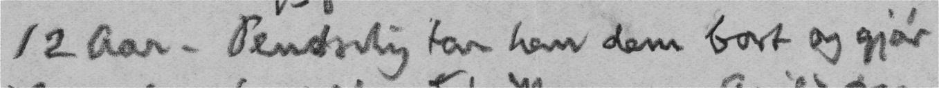12 Aar. Pludselig tar han dem bort og gjør
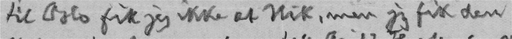til Oslo fik jeg ikke et Nik, men jeg fik den
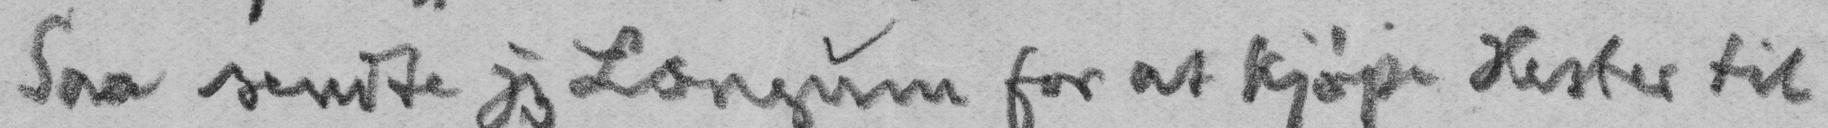Saa sendte jeg Longum for at kjøpe Hester til
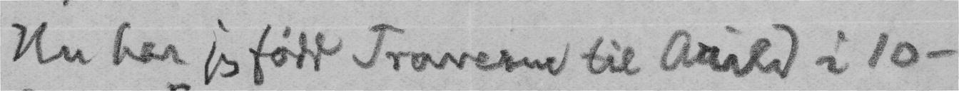Nu har jeg fødd Traverne til Arild i 10-
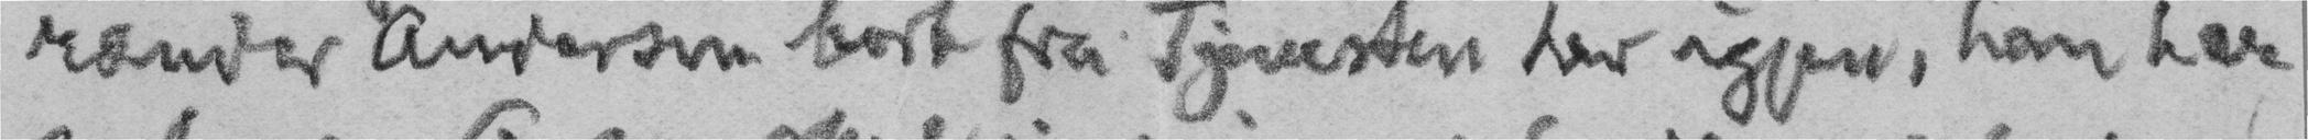rænder Andersen bort fra Tjenesten her igjen, han har
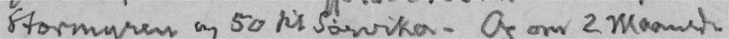Stormyren og 50 til Sørvika. Og om 2 Maaneder
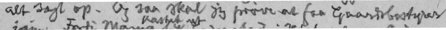alt sagt op. Og saa skal jeg prøve at faa Gaardsbestyrer
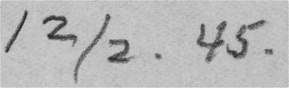12/2. 45.
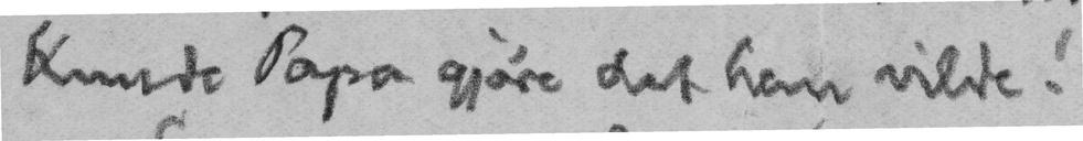kunde Papa gjøre det han vilde!
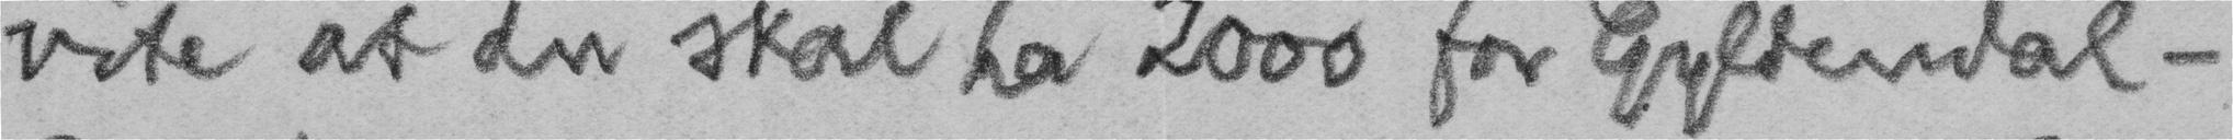vite at du skal ha 2000 for Gyldendal-
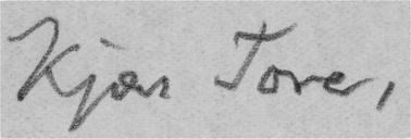Kjære Tore,
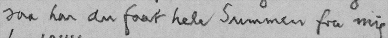saa har du faat hele Summen fra mig
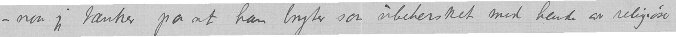– naar jeg tænker paa at han bryter saa ubehersket med hende av religiøse
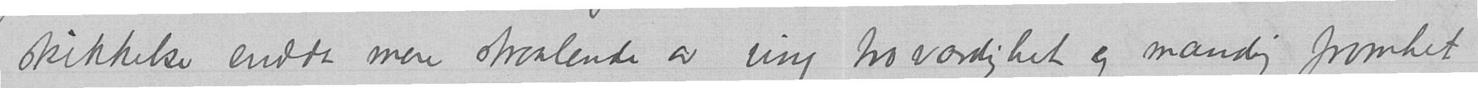skikkelse endda mere straalende av ung troværdighet og mandig fromhet
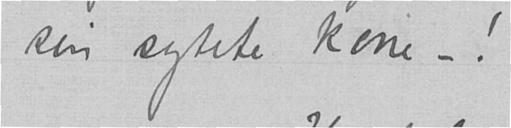sin sytete kone –!
